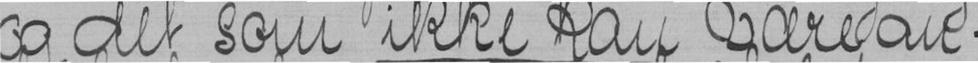og det som ikke kan være an-
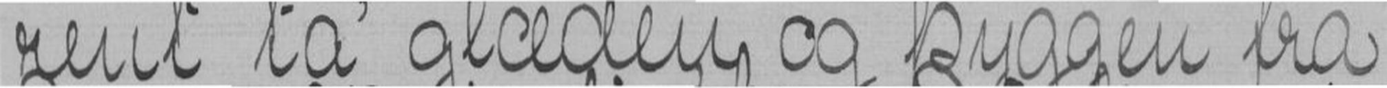rent la glæden og hyggen fra
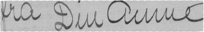fra Din Annie
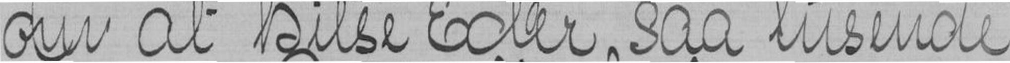om at hilse Eder saa tusende
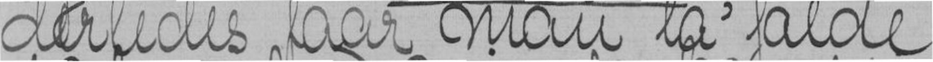derledes faar man la' falde.
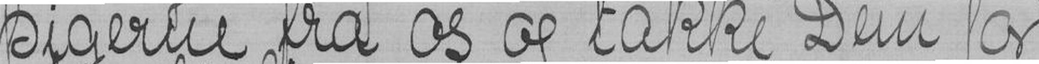pigerne fra os og takke Dem for
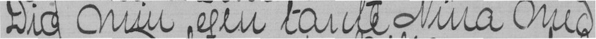Dig min egen tante Nina med
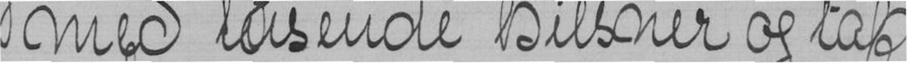med tusende hilsner og tak
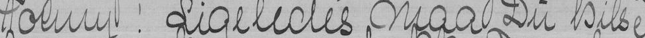Tonny! Ligeledes maa Du hilse
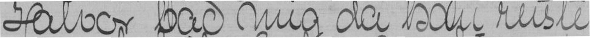Halvor bad mig da han reiste
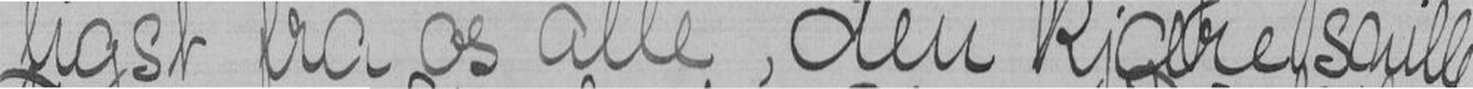ligst fra os alle, den kjære snille
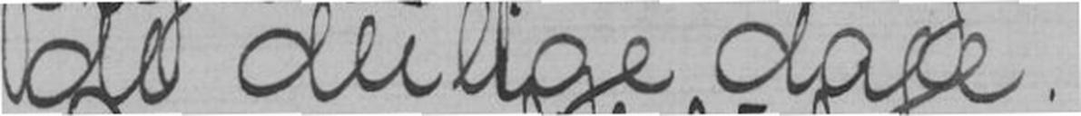de deilige dage.
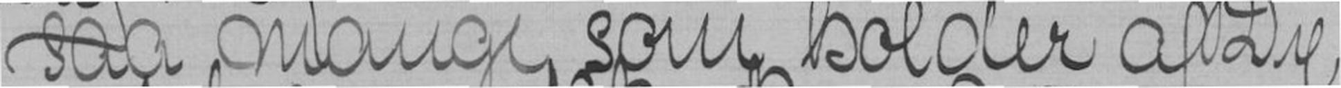saa mange som holder af Dig,
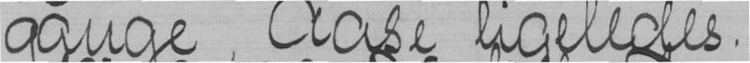gange, Aase ligeledes.
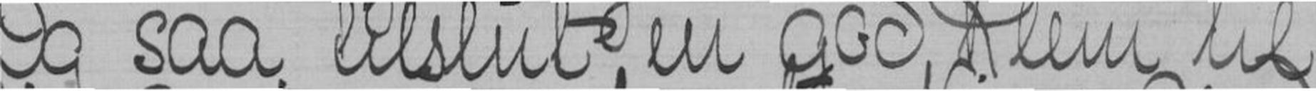Og saa tilslut en god Klem til
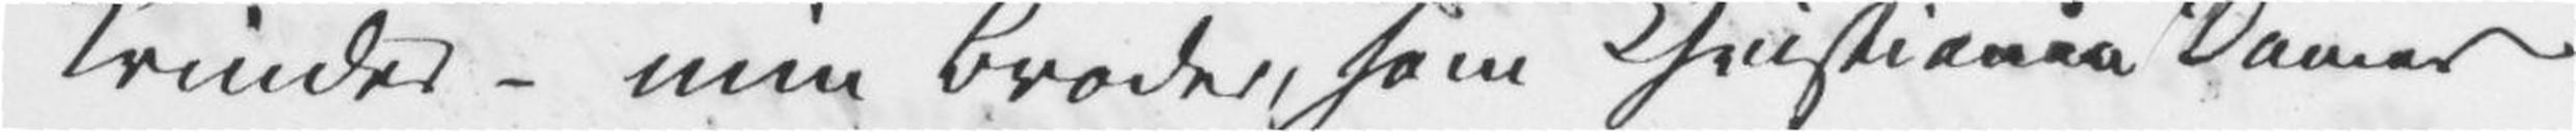Kvinder» – min Broder, som Christiania Damer
